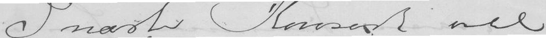I næste Konsert vil
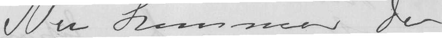Nu kommer Du
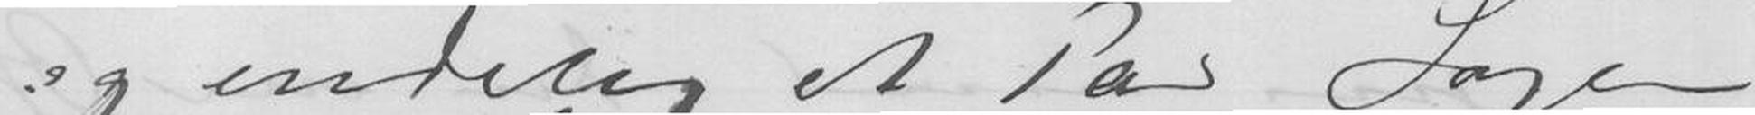og endelig et Par Sager
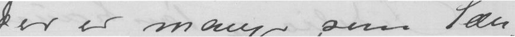der er mange som Læn-
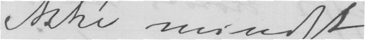ikke mindst
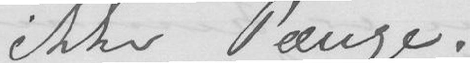ikke Pænge.
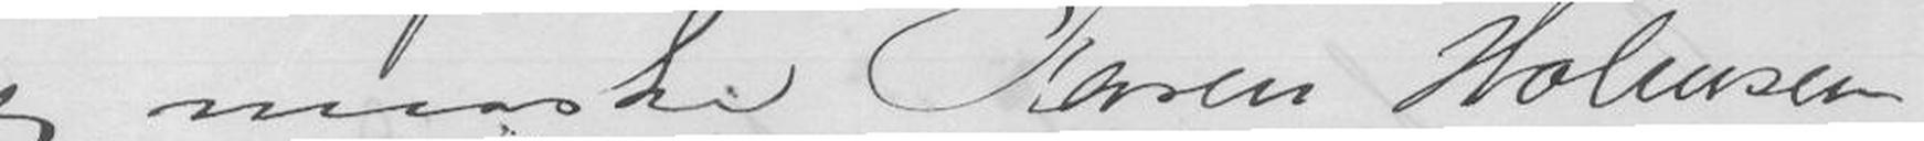og maaske Karen Holmsen
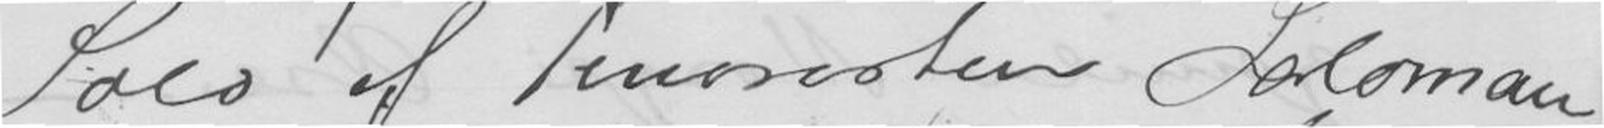Solo af Tenoristen Saloman
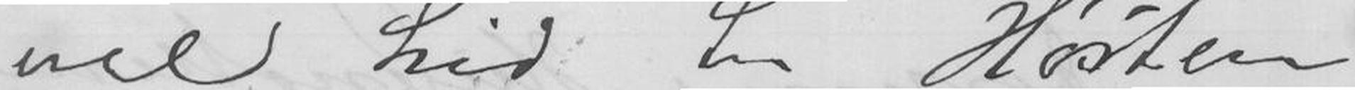vel hid til Høsten
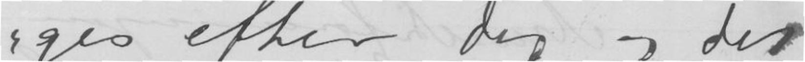ges efter Dig og Dit
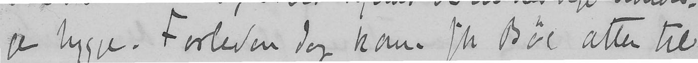ge hygge. Forleden dag kom frk Bøe atter til
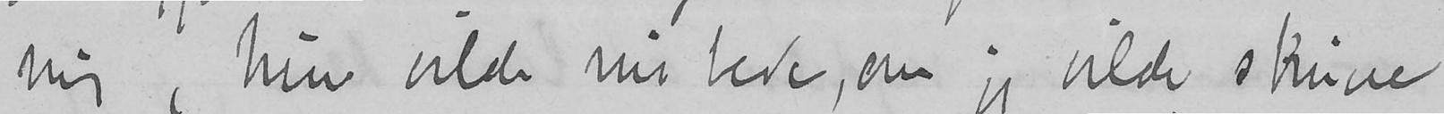mig, hun vilde nu bede, om jeg vilde skrive
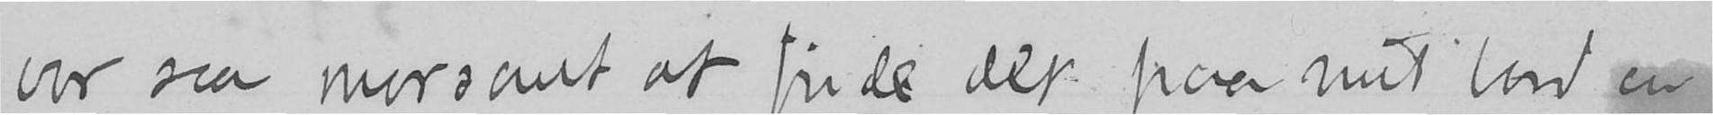var saa morsomt at finde det paa mit bord en
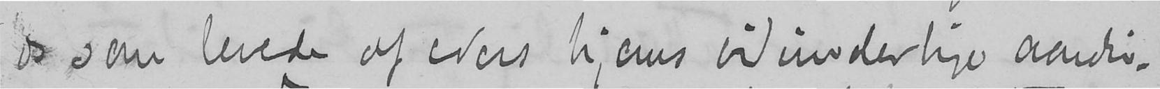os som levede af eders hjems vidunderlige aandri-
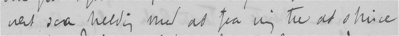vært saa heldig med at faa mig til at skrive
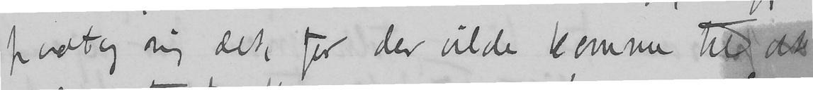paatog mig det, for der vilde komme til at
